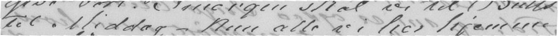til Middag - kun alle vi her hjemme
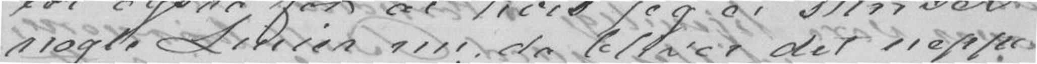nogle Linier nu da bliver det neppe
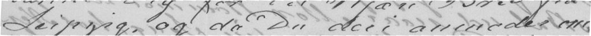Leipzig, og da Du deri anmoder om.
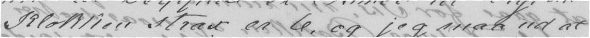Klokken strax er 6, og jeg maa ud at
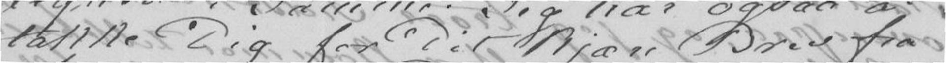takke Dig for Dit kjære Brev fra
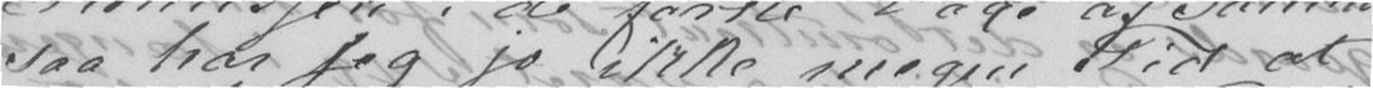saa har jeg jo ikke megen Tid at
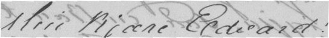Min kjære Edvard!
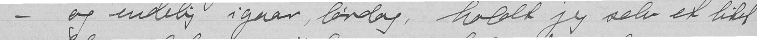og endelig igaar, lørdag, holdt jeg selv et lidet
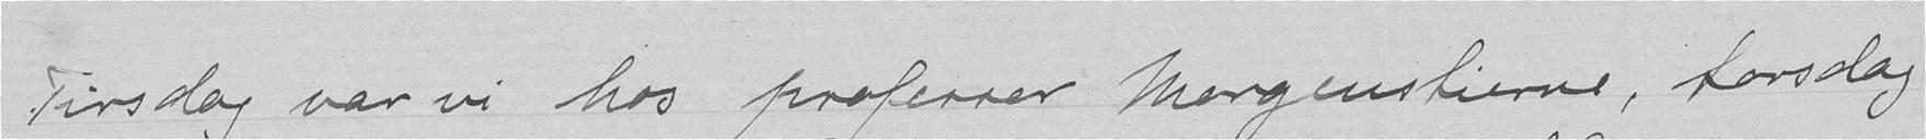Tirsdag var vi hos professor Morgenstierne, torsdag
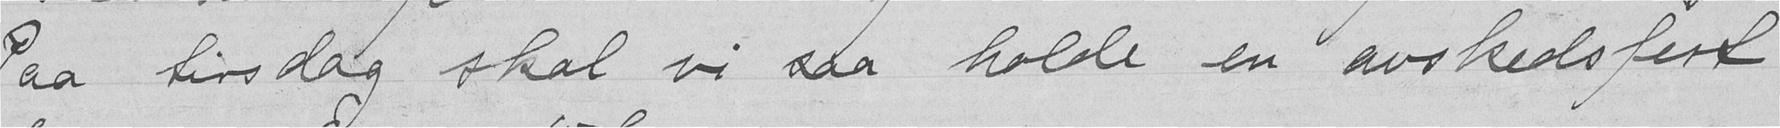Paa tirsdag skal vi saa holde en avskedsfere
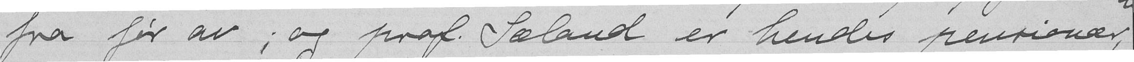fra før av ; og prof. Sæland er hendes pentionær,
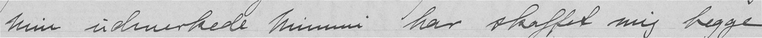min udmerkede Ninni har skaffet mig begge
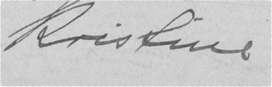Kristine
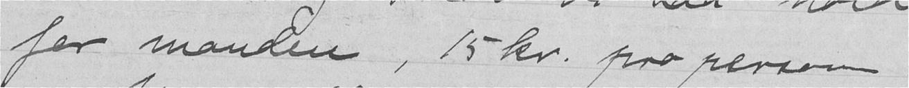for manden, 15 kr. pro person
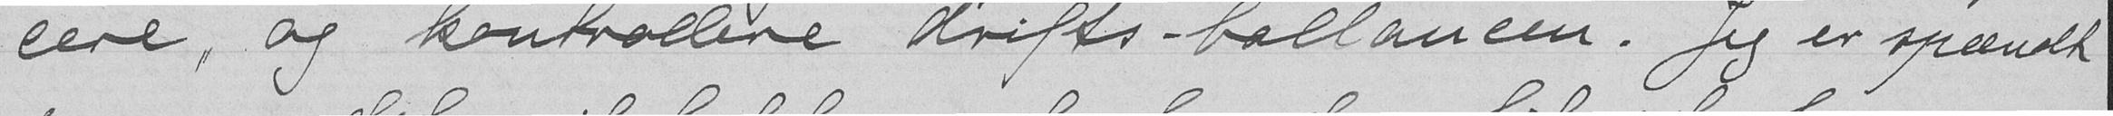cere, og kontrollere drifts-ballancen. Jeg er speendk
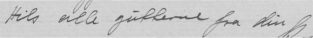Hils alle gufterne fra den
